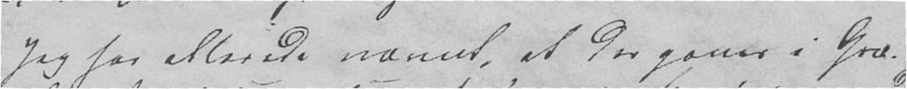Jeg har allerede nævnt, at der gaves i Græ-
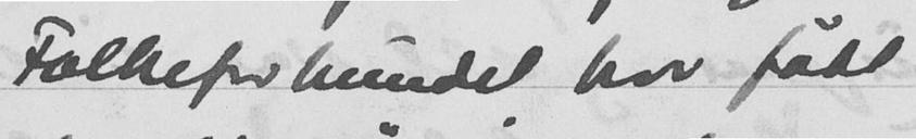Folkeforbundet har fått
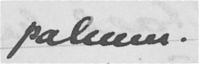palinen.
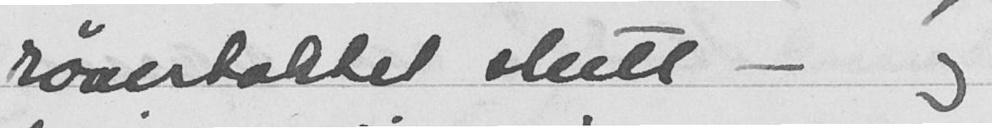røvertoktet slutt - og
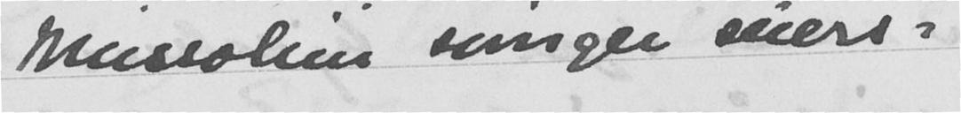Mussolini ringer siste-
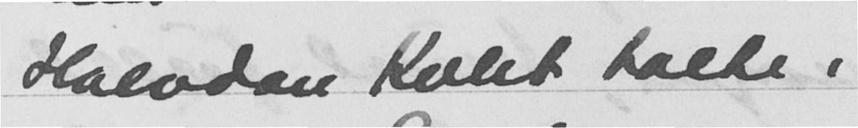Halvdan Koht talte i
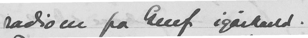radioen fra Genf igårkveld.
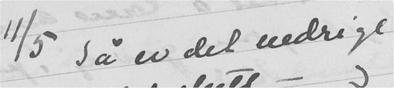11/5 Så er det nedrige
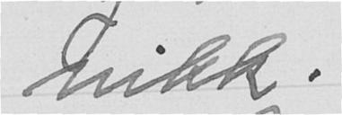nikk.
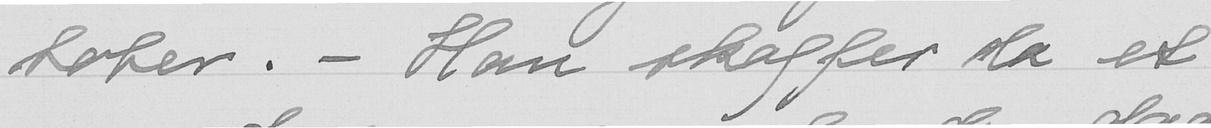tober. - Han skaffer da et
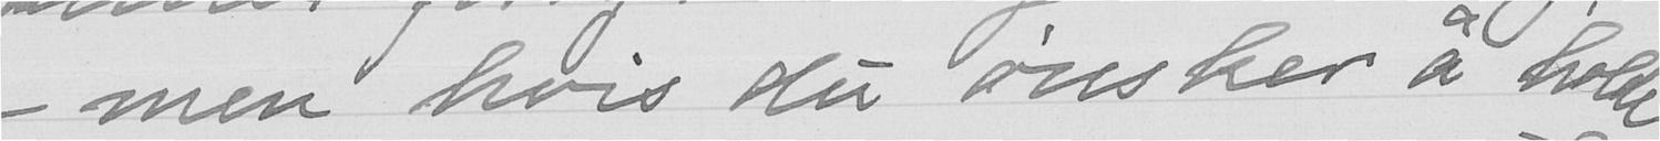- men hvis du ønsker å holde
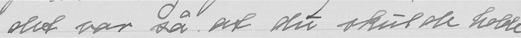det var så at du skulde holde
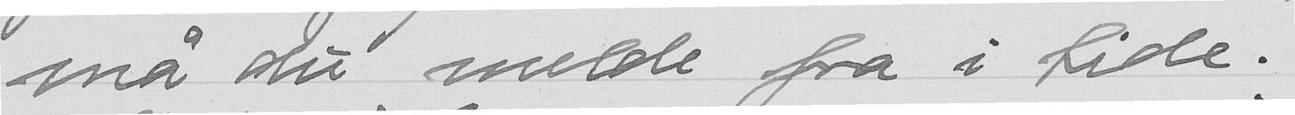må du melde fra i tide.
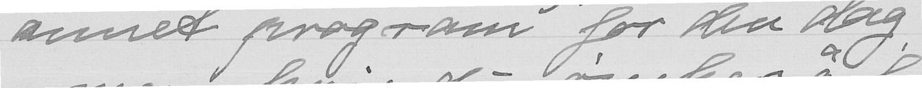annet program for den dag,
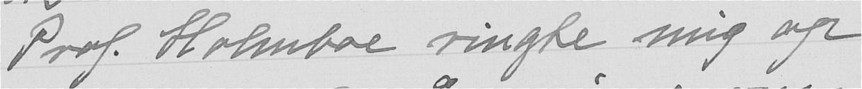Prof. Holmboe ringte mig op
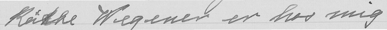Käthe  Wegener er hos mig
